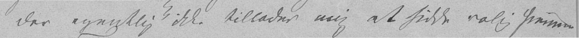der egentlig ikke tillader mig at sidde rolig hjemme
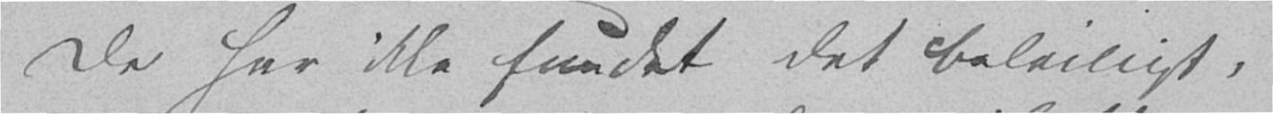De har ikke fundet det beleiligt,
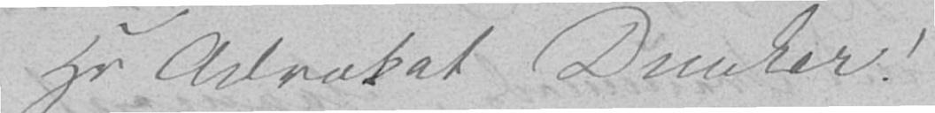Hr Advokat Dunker!
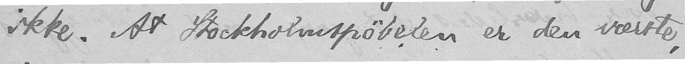ikke. At Stockholmspøbelen er den værste,
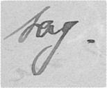sag.
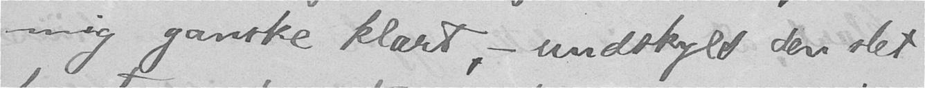mig ganske klart, – undskyld den det
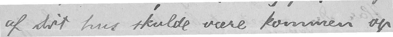af dit hus skulde være kommen op
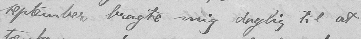september bragte mig daglig til at
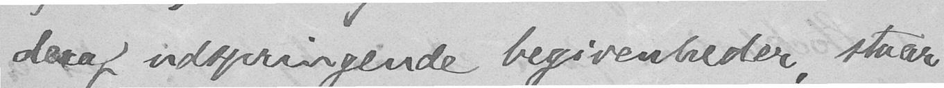deraf udspringende begivenheder, staar
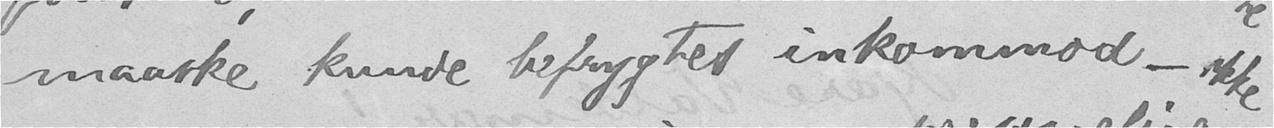maaske kunde befrygtes inkommod - ikke
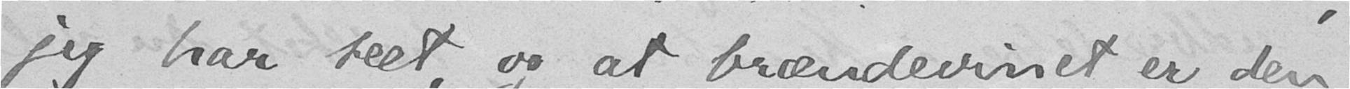jeg har seet, og at brændevinet er den
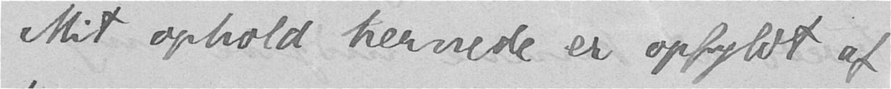Mit ophold hernede er opfyldt af
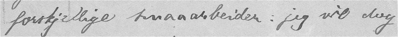forskjellige smaaarbeider: jeg vil dog
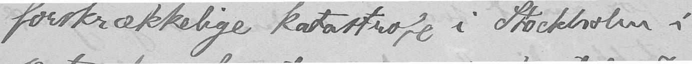forskrækkelige katastrofe i Stockholm i
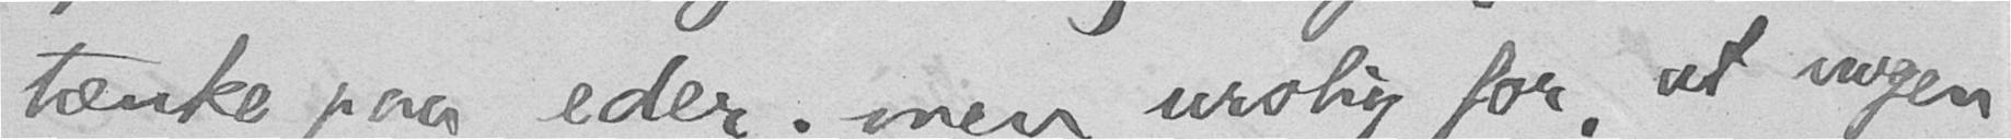tænke paa eder; men urolig for, at nogen
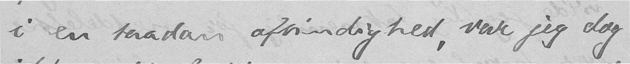i en saadan afsindighed, var jeg dog
